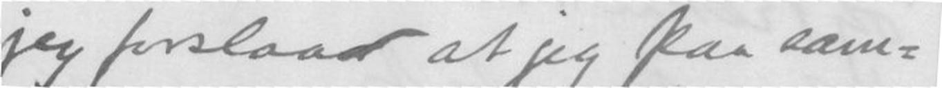jeg forstaa at jeg paa sam-
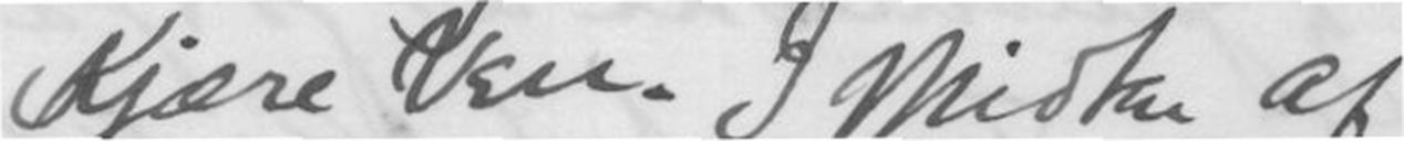Kjære Ven. I Midten af
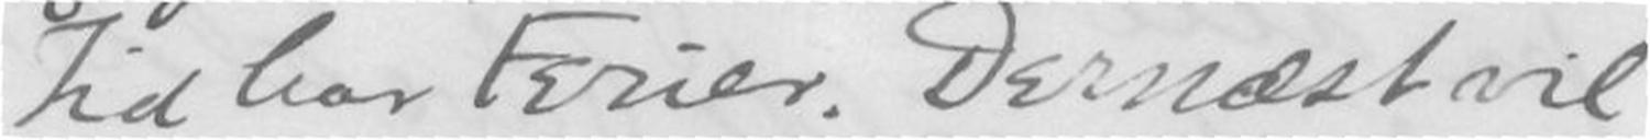Tid har Ferier. Dernæst vil
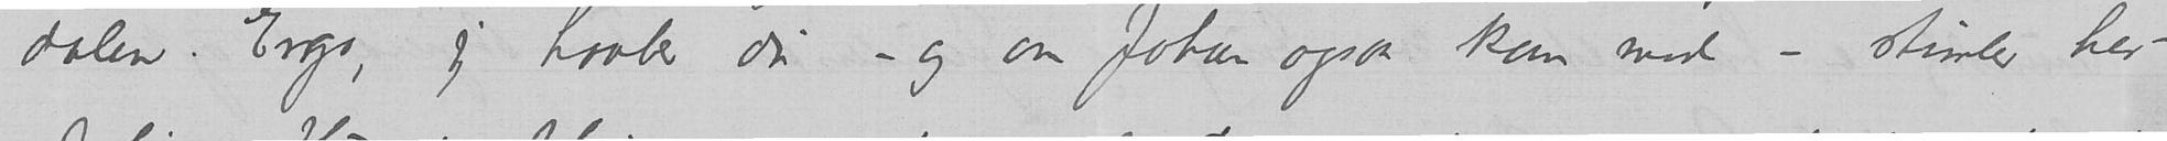dalen. Ergo, jeg haaber du – og om Johan ogsaa kon med – stimler her-
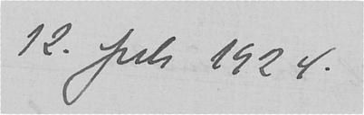12 . juli 1924.
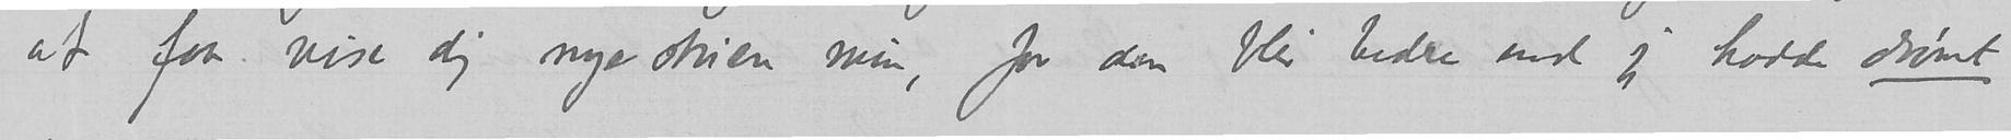at faa vise dig nye stuen min, for den blir bedre end jeg hadde drømt
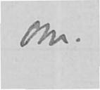om.
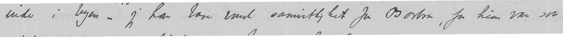inde i byen – jeg har bare vond samvittighet for Barbra, for hun var saa
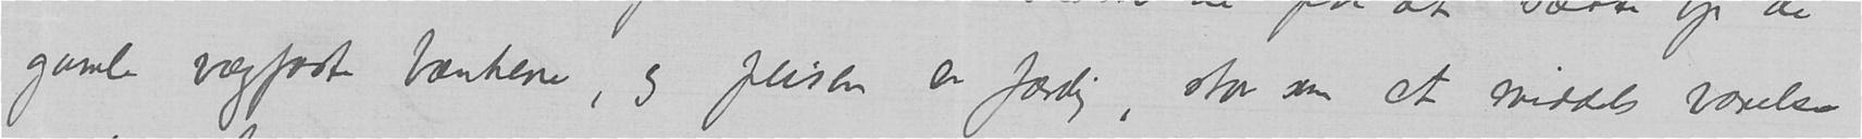gamle vægfaste bænkene, og peisen er færdig, stor som et middels værelse
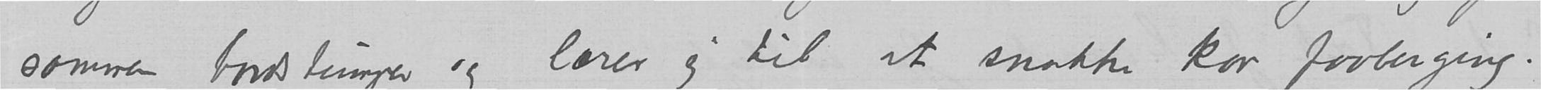sammen bordstumper og lærer sig til at snakke kan faaberging.
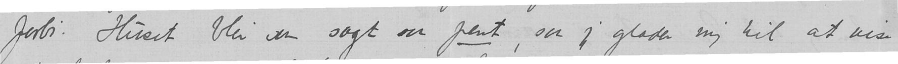forbi. Huset blir som sagt saa pent, saa jeg glæder mig til at vise
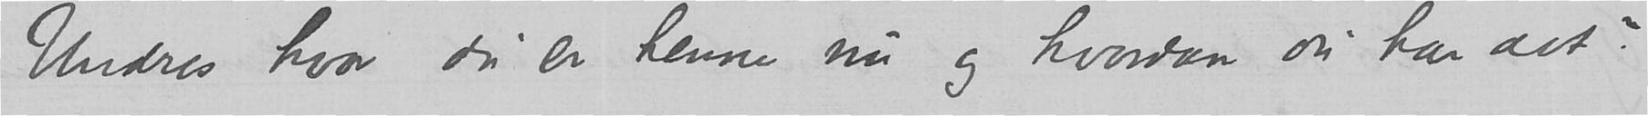Undres hvor du er henne nu og hvordan du har det?
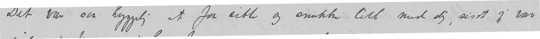Det var saa hyggelig at faa sitte og snakke litt med dig, sisst jeg var
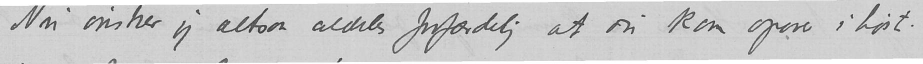Nu ønsker jeg altsaa aldeles forfærdelig at du kom opover i høst.
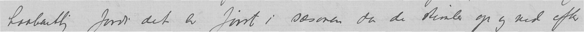haabentlig fordi det er først i sæsonen da de stimler op og ved efter
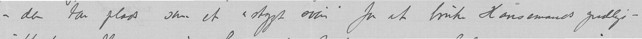– den tar plads som et – «stygt svin» for et bruke Hansemands yndlings-
